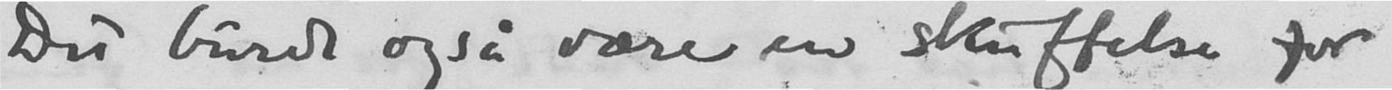Det burde også være en skuffelse for
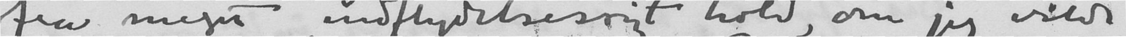fra meget indflydelsesrigt hold, om jeg vilde
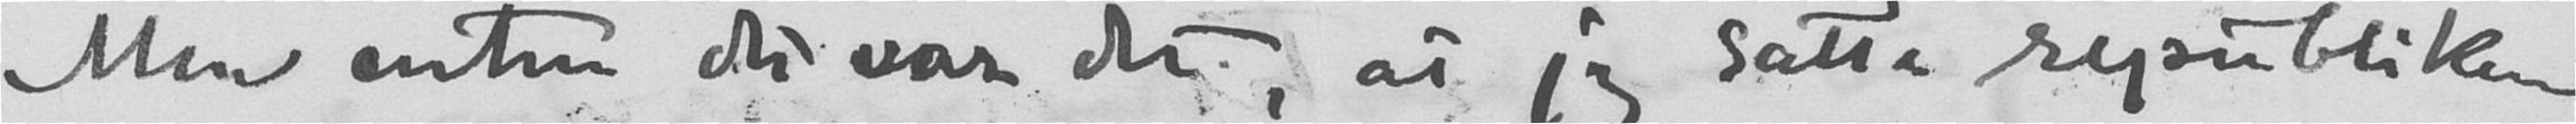Men enten det var det, at jeg satte republiken
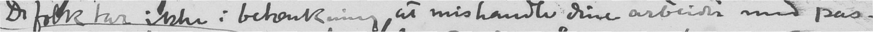De folk tar ikke i betænkning at mishandle dine arbeider pas-
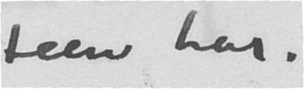teen har.
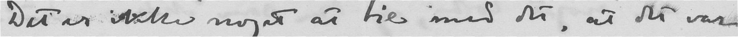Det er ikke noget at tie med det, at det var
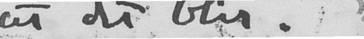at det blir.
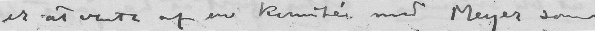er at vente af en komité Med Meyer som
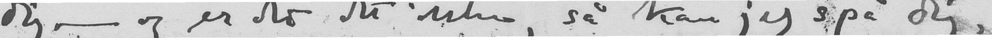dig - og er det det ikke, så kan jeg spå dig,
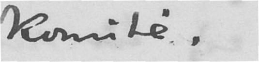komité.
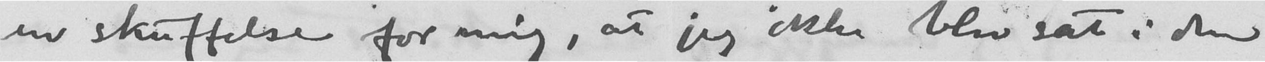en skuffelse for mig, at jeg ikke blev sat i den
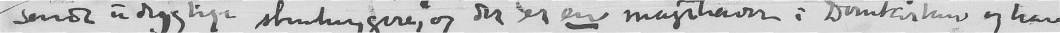sende udygtige stenhuggere, og der er en magthaver i Domkirken og han
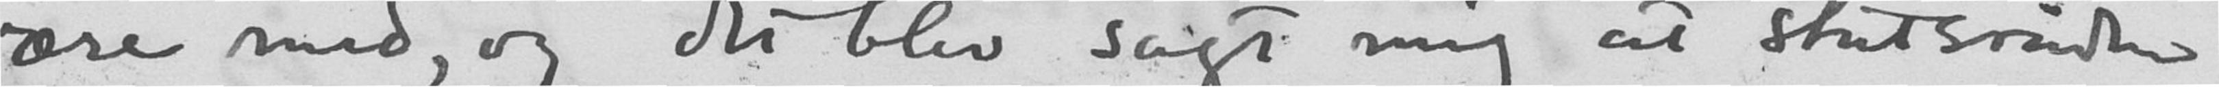være med, og det blev sagt mig at statsråden
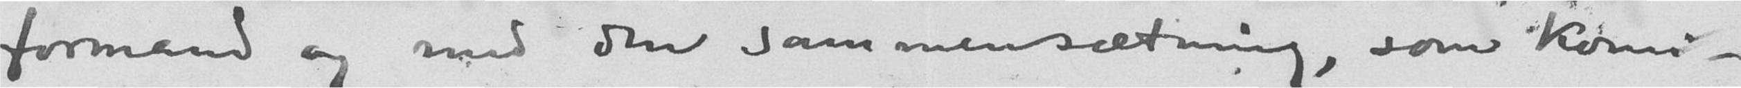formand og med den sammensætning, som komi-
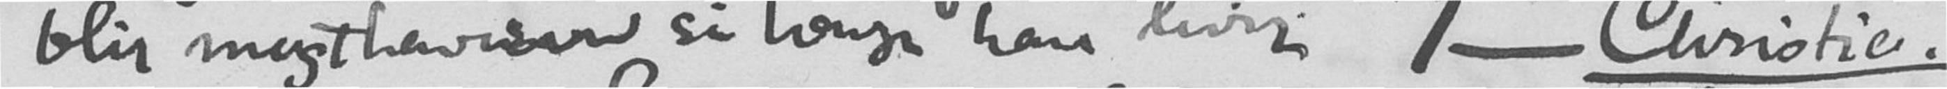blir magthaveren så længe han lever - Christie.
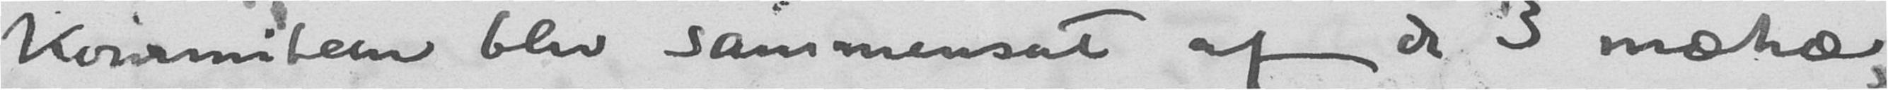komiteen blev sammensat af de 3 mæhæ,
